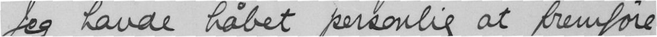Jeg havde håbet personlig at fremføre
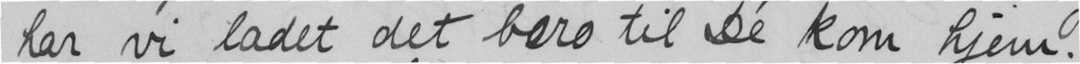har vi ladet det bero til De kom hjem.
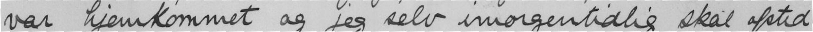var hjemkommet og jeg selv imorgentidlig skal afsted
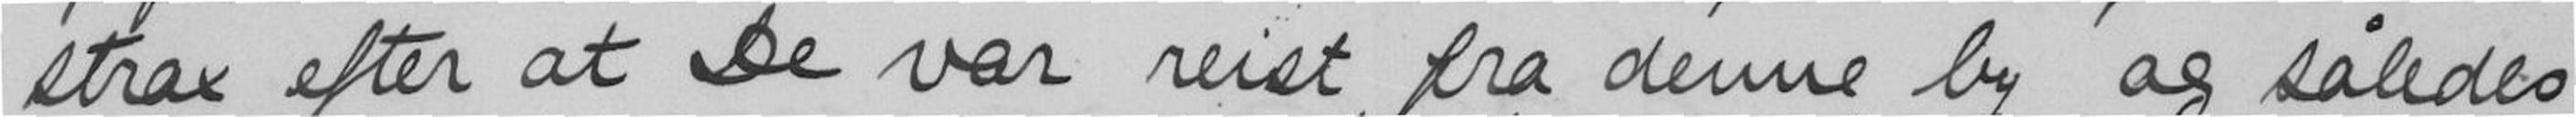strax efter at De var reist fra denne by og således
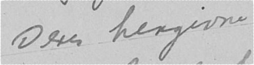Din hengivne
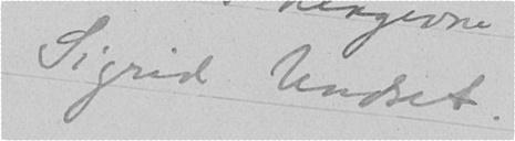Sigrid Undset.
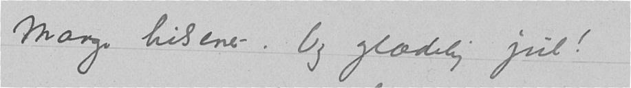Mange hilsener -. Og glædelig jul!
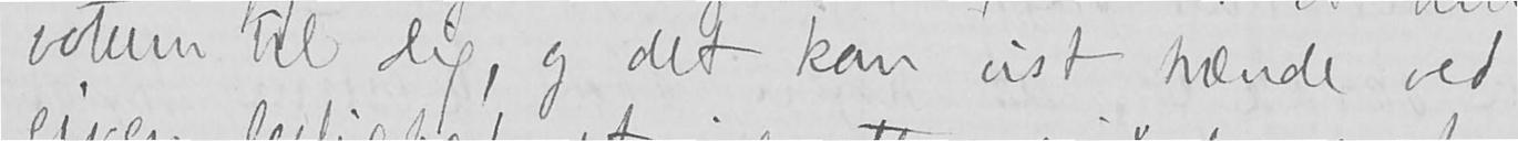votum til Dig, og det kan vist hænde ved
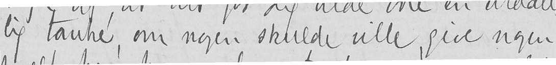lig tanke, om nogen skulde ville give nogen
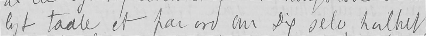lyt taale et par ord om Dig selv, hvilket
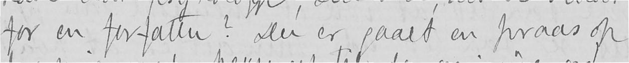for en forfatter? Der er gaaet en praas op
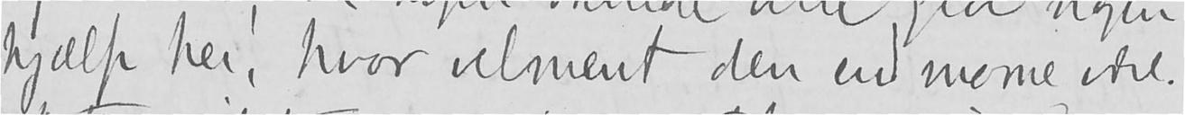hjælp her, hvor velment den end monne være.
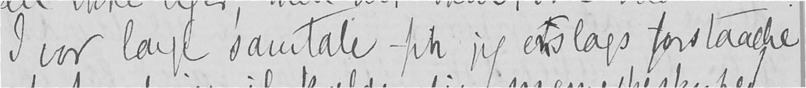I vor lange samtale fik jeg enslags forstaaelse
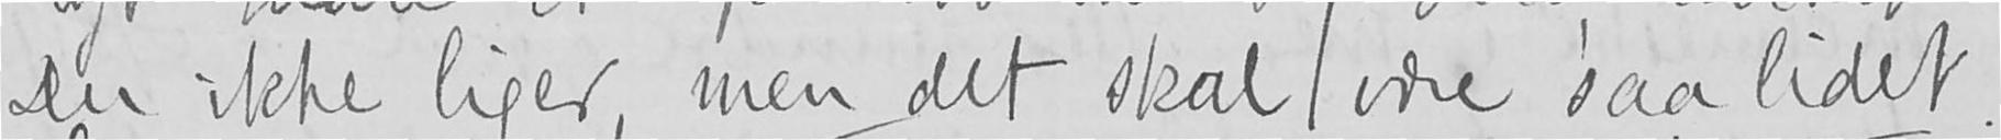Du ikke liger, men det skal være saa lidet.
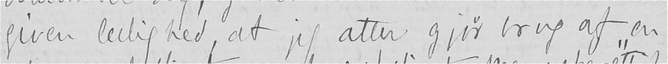given leilighed, at jeg atter gjør brug af en
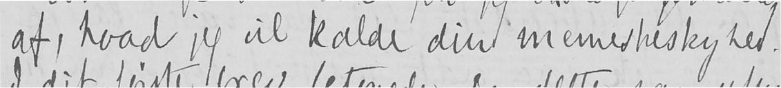af, hvad jeg vil kalde din menneskeskyhed.
